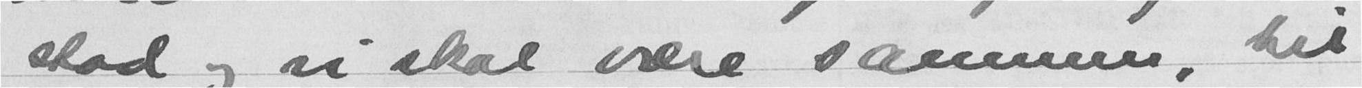stad og vi skal være sammen, til
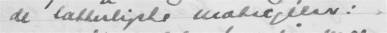de latterligste motsigelser!
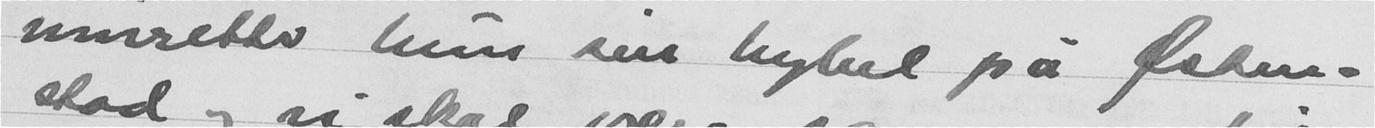innsetter hun sin hybel på Østen-
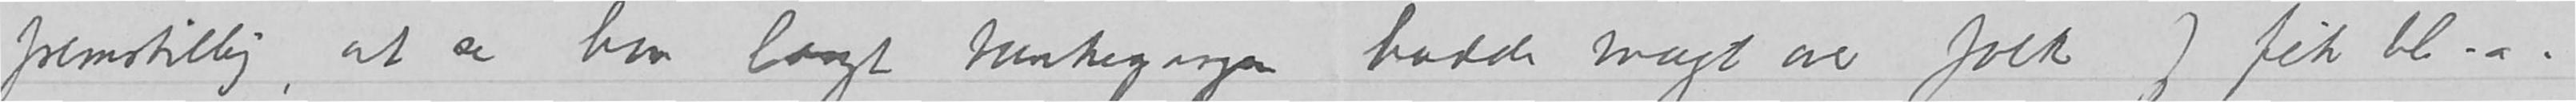fremstilling, at se hvor langt tankegangen hadde magt over folk Jeg fik bl.a.
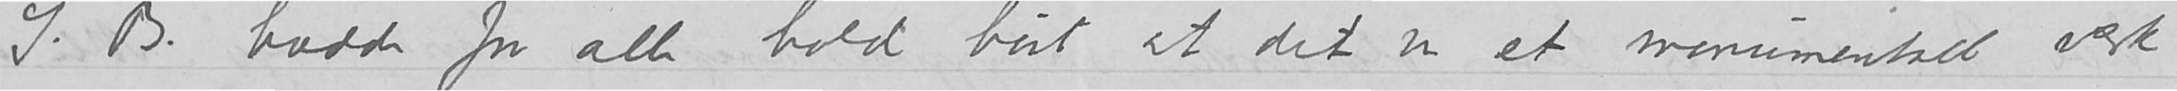S.B. hadde fra alle hold hørt at det er et monumentalt værk,
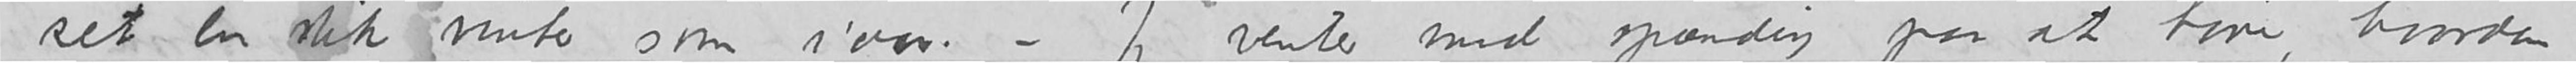set en slik vinter som iaar. - Jeg venter med spænding paa at høre, hvordan
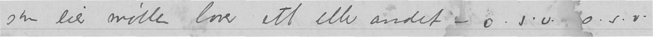som eier møllen lover ett eller andet -o.s.v. o.s.v.
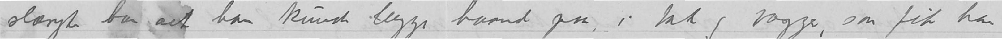slængte han alt han kunde legge haand paa, i tak og vægger, saa fik
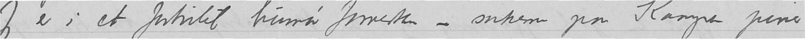Jeg er i et fortvilet humør forresten - sakerne paa Kampen piner
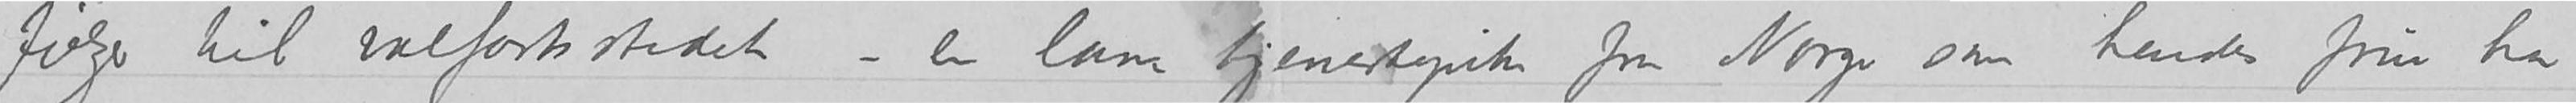følger til valfartsstedet - en lam tjenestepike fra Norge som hendes frue har
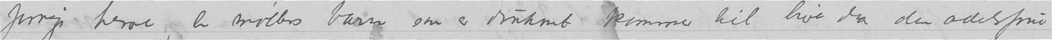forrige herre, en møllers barn som er druknet kommer til live da den adelsfrue
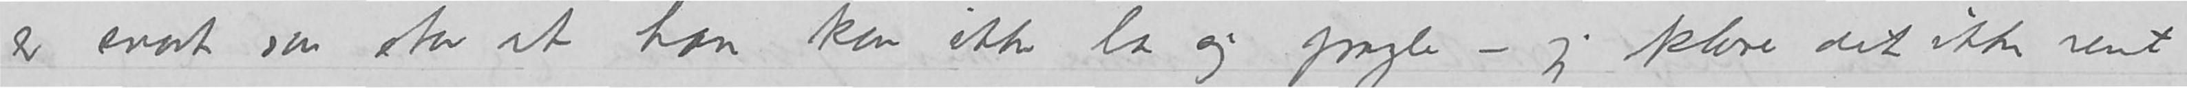er snart saa stor at han kan ikke la sig pryle - jeg klarer det ikke rent korporlig.
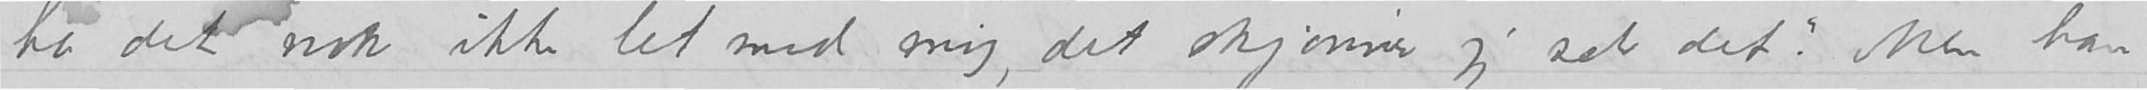har det nok ikke let med mig, det skjønner jeg selv det.» Men han
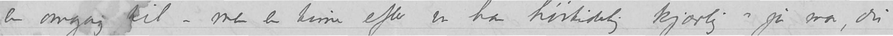han en omgang til - men en time efter var han høitidelig kjærlig «ja mor, du
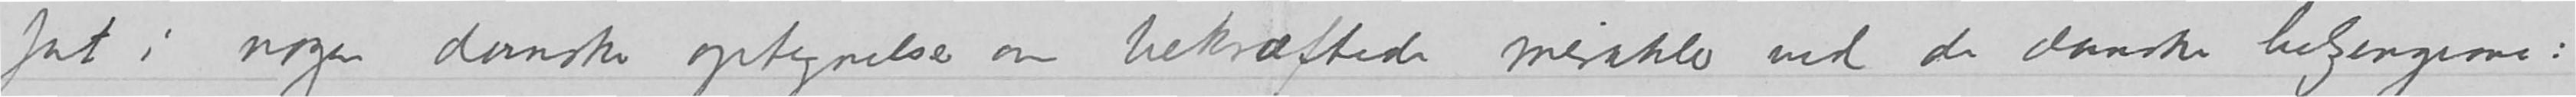fat i nogen danske optegnelser om bekræftede mirakler ved de danske helgengerne:
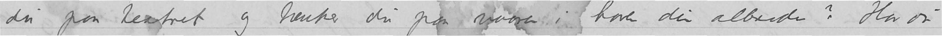du paa teatret og tænker du på vaaren i haven din allerede? Har vi
